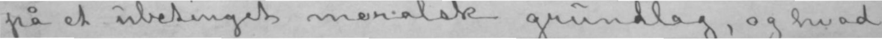på et ubetinget moralsk grundlag, og hvad
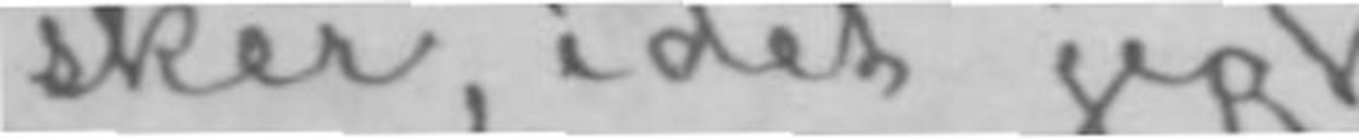sker, idet jeg
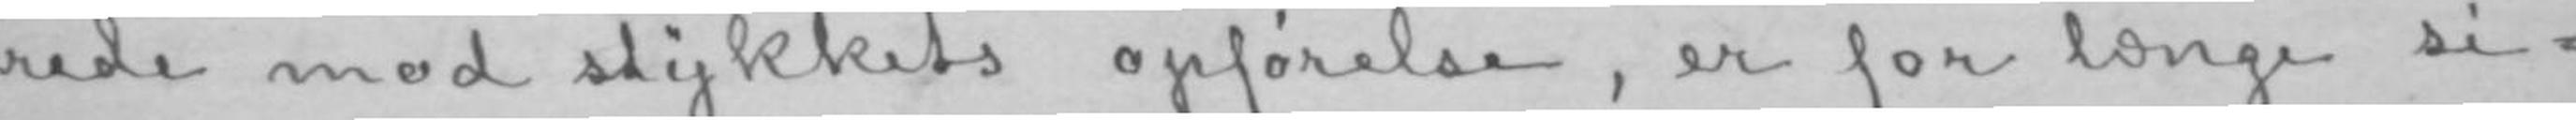rede mod stykkets opførelse, er for længe si-
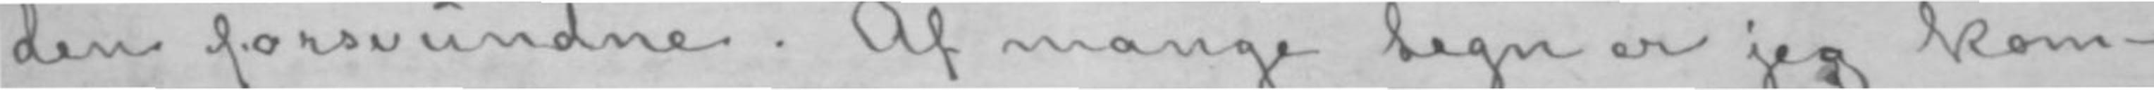den forsvundne. Af mange tegn er jeg kom-
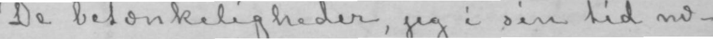De betænkeligheder, jeg i sin tid næ-
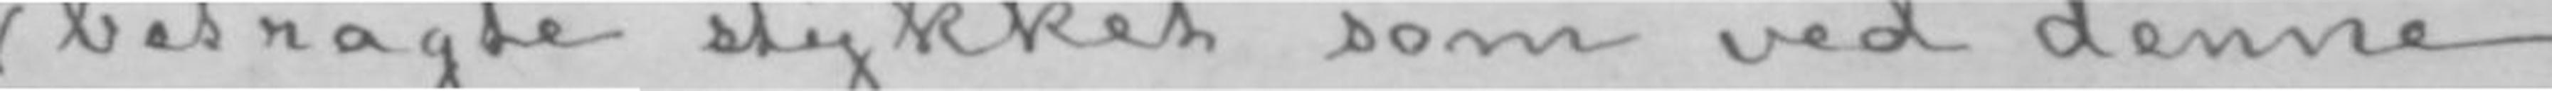betragte stykket som ved denne
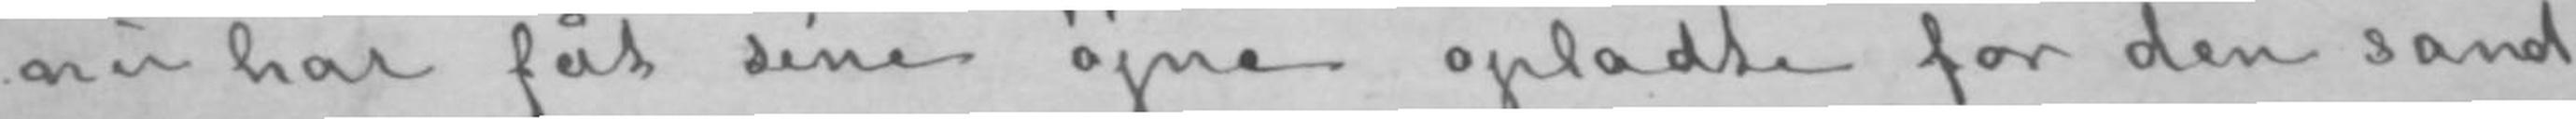nu har fåt sine øjne opladte for den sand-
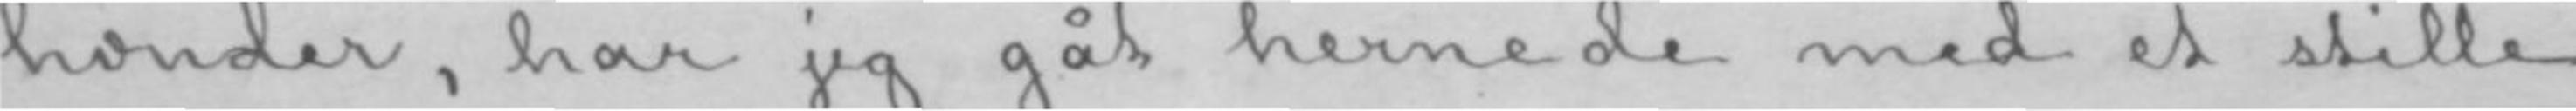hænder, har jeg gåt hernede med et stille
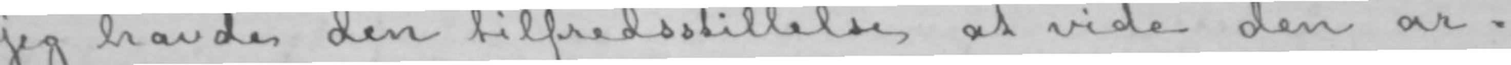jeg havde den tilfredsstillelse at vide den ar-
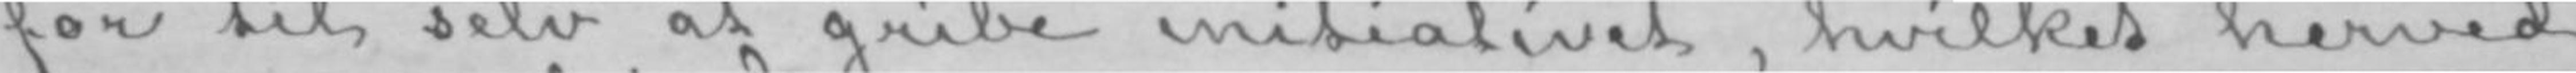for til selv at gribe initiativet, hvilket herved
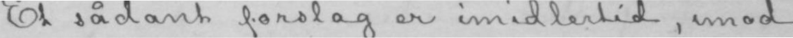Et sådant forslag er imidlertid, imod
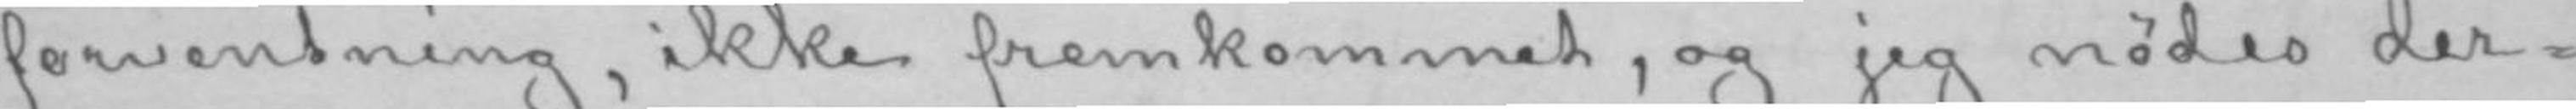forventning, ikke fremkommet, og jeg nødes der-
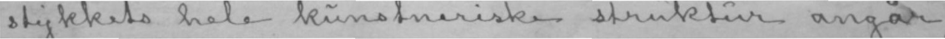stykkets hele kunstneriske struktur angår,
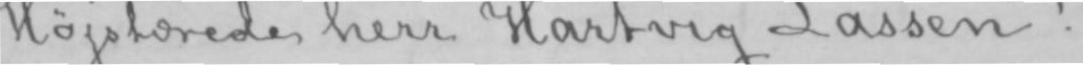Højstærede herr Hartvig Lassen!
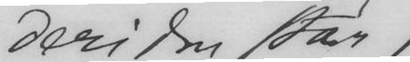deri den står)
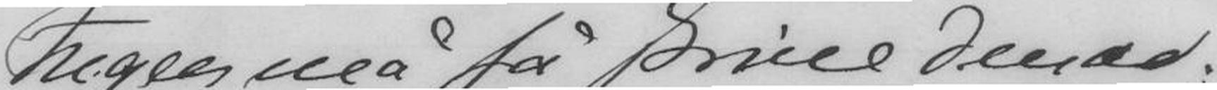(Ingen må få skrive den av;
